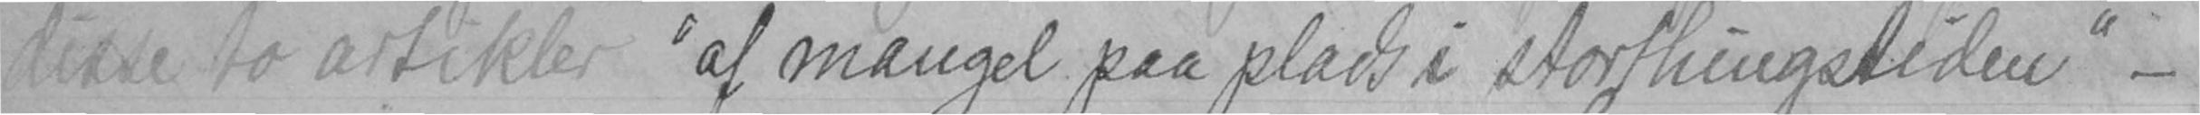disse to artikler "af mangel paa plads i storthingstiden" -
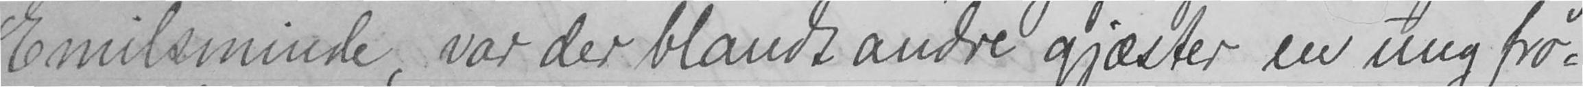Emilsminde, var der blandt andre gjæster en ung frø-
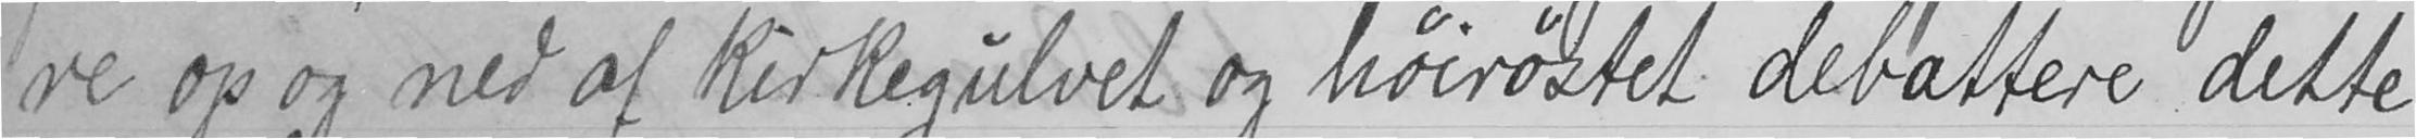re op og ned af kirkegulvet og høirøstet debattere dette
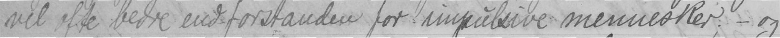vel ofte bedre end forstanden for impulsive mennesker; - og
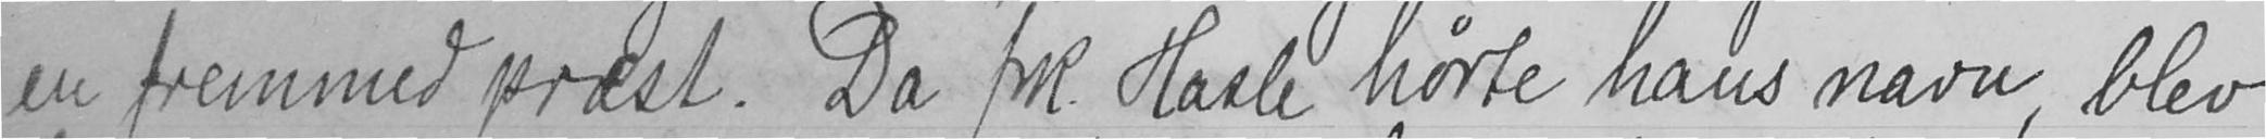en fremmed præst. Da frk. Hasle hørte hans navn, blev
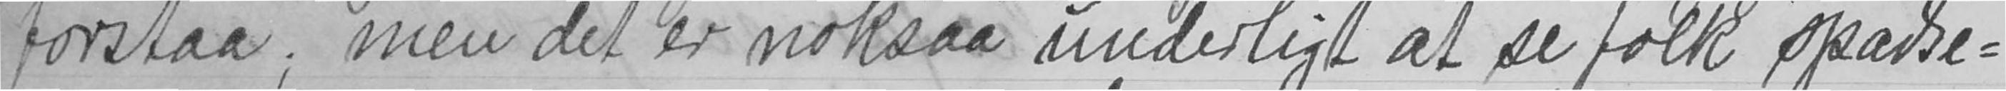forstaa; men det er noksaa underligt at se folk spadse-
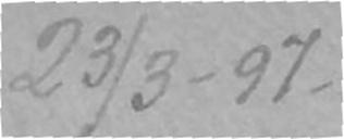23/3-97.
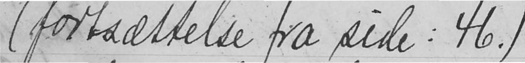(fortsættelse fra side: 46.)
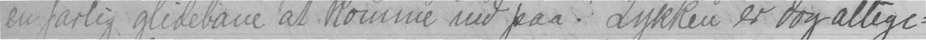en farlig glidebane at komme ind paa! Lykken er dog allige-
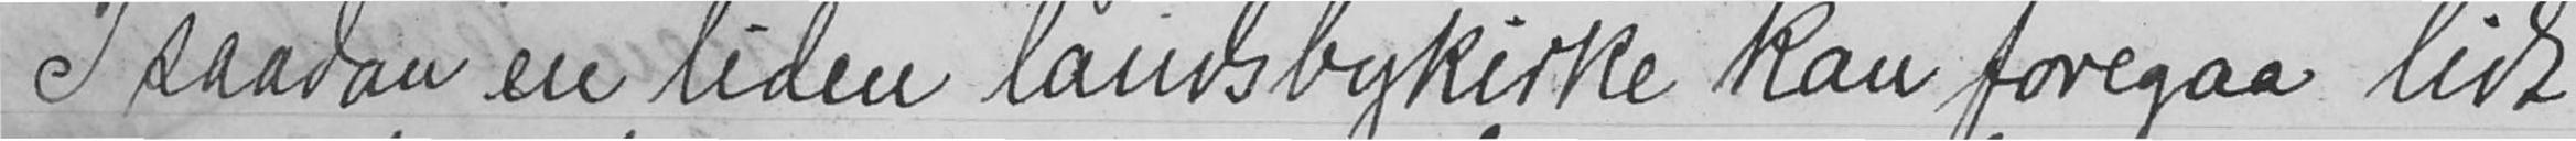I saadan en liden landsbykirke kan foregaa lidt
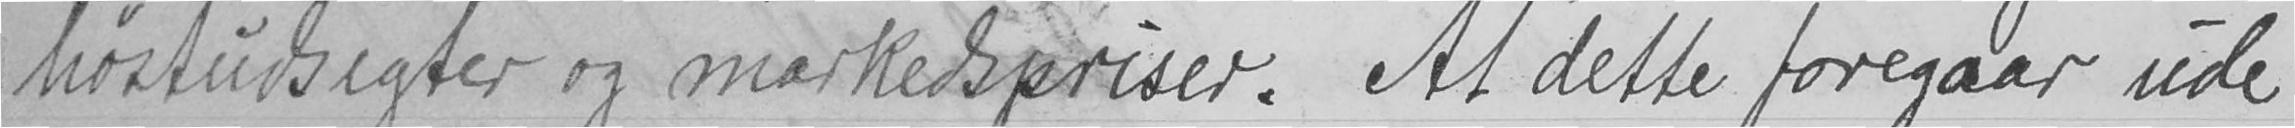høstudsigter og markedspriser. At dette foregaar ude
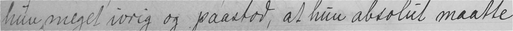hun meget ivrig og paastod, at hun absolut maatte
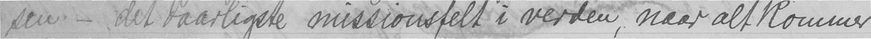sen - det daarligste missionsfelt i verden, naar alt kommer
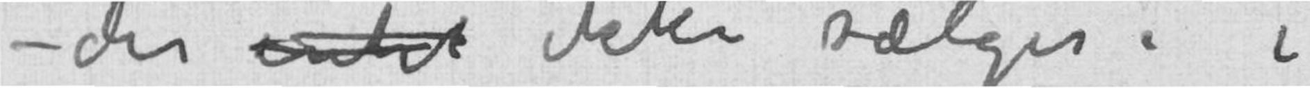derikke sælges i
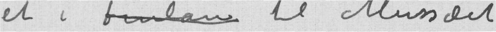et i Finlan til Musæet
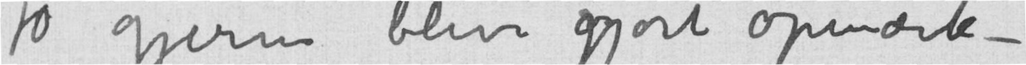jo gjerne blive gjort opmærk-
